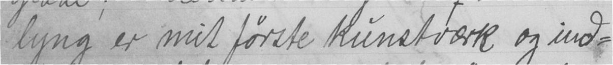lyng er mit første kunstværk og ind-
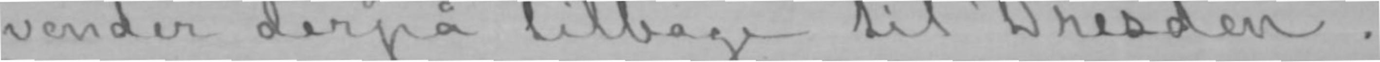vender derpå tilbage til Dresden.
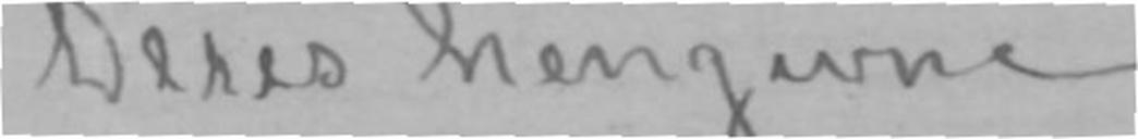Deres hengivne
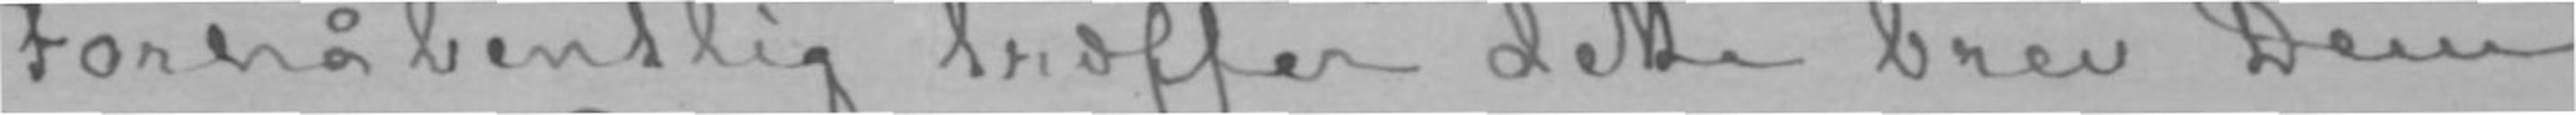Forhåbentlig træffer dette brev Dem,
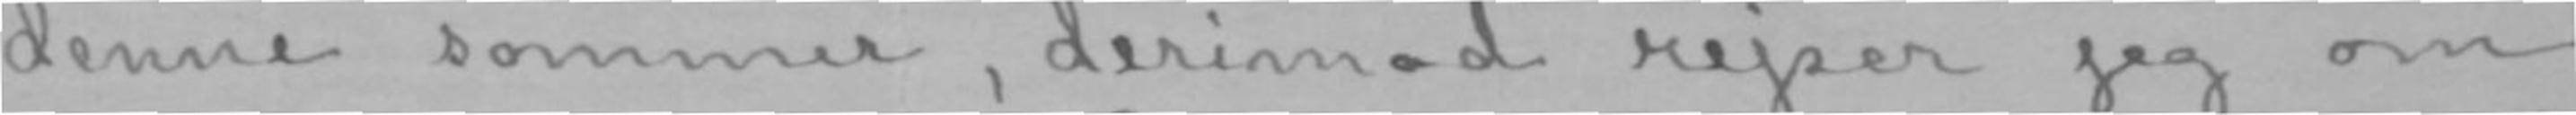denne sommer, derimod reiser jeg om
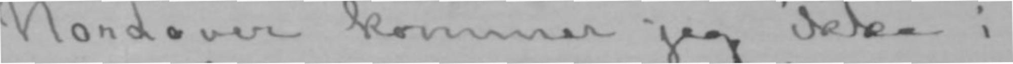Nordover kommer jeg ikke i
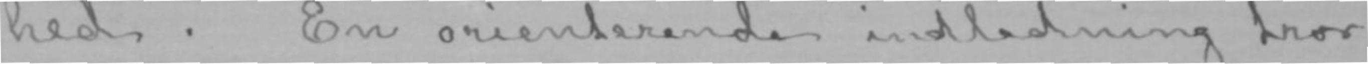hed. En orienterende indledning tror
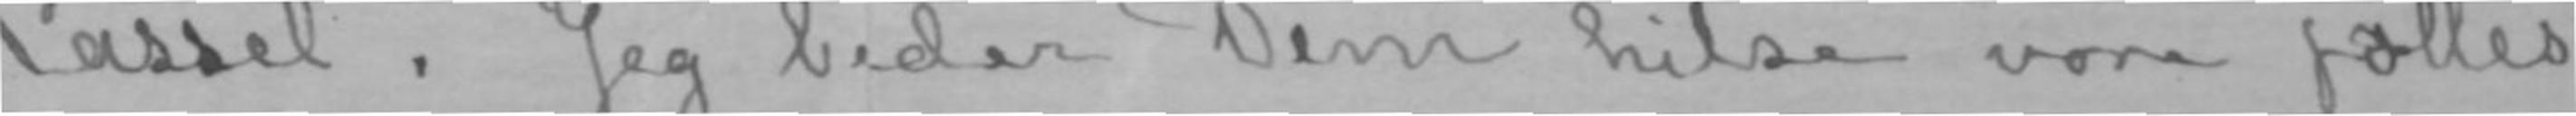Cassel. Jeg beder Dem hilse vore fælles
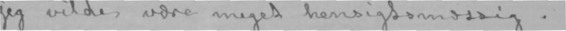jeg vilde være meget hensigtsmæssig.
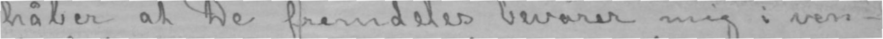håber at De fremdeles bevarer mig i ven-
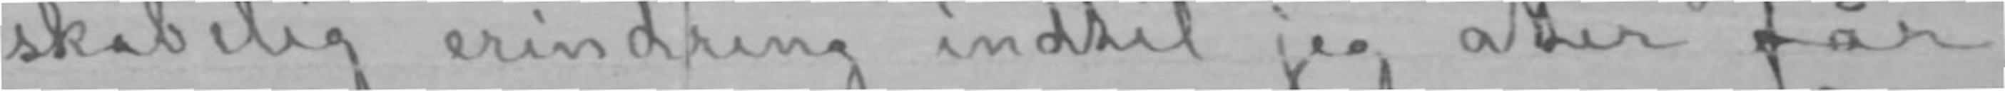skabelig erindring indtil jeg atter får
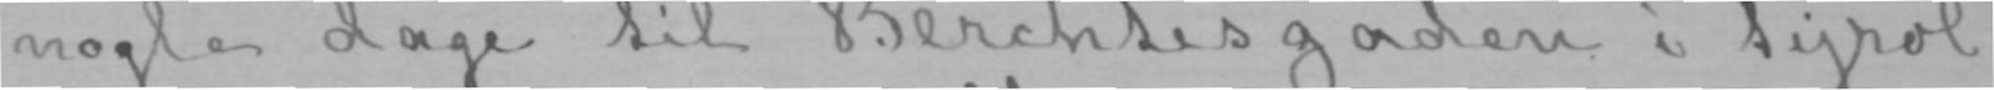nogle dage til Berchtesgaden i Tyrol,
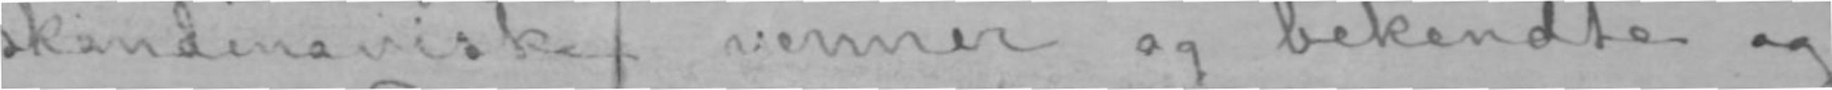skandinaviske venner og bekendte og
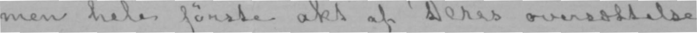men hele første akt af Deres oversættelse
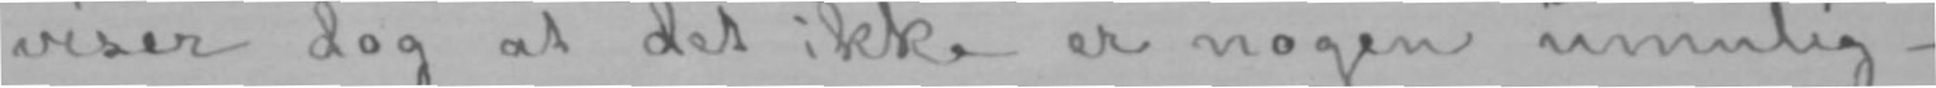viser dog at det ikke er nogen umulig-
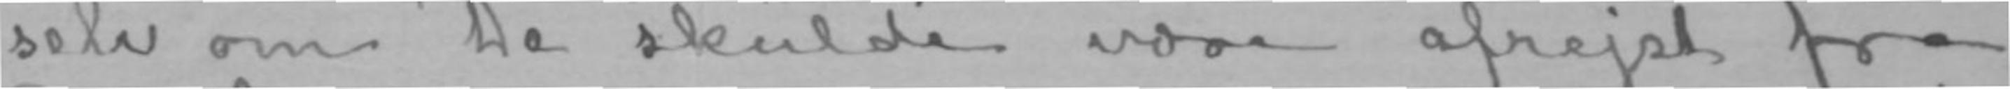selv om De skulde være afrejst fra
